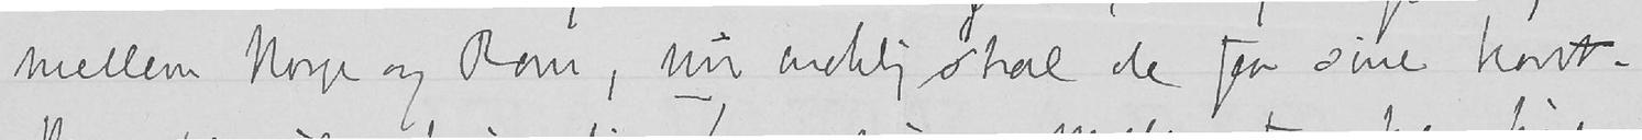mellem Norge og Rom, nu endelig skal de faa sine kort.
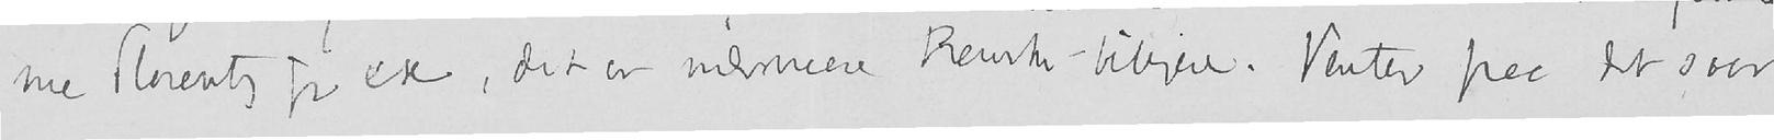me Florenz for ex, det er nærmere kanske billigere. Venter paa det svar
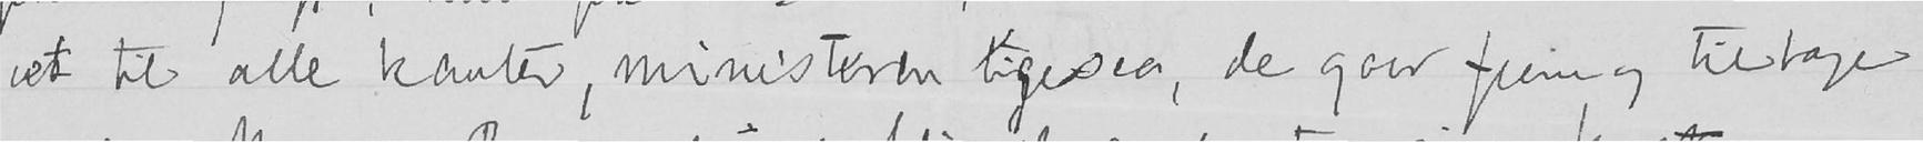vet til alle kanter, ministeren ligesaa, de gaar frem og tilbage
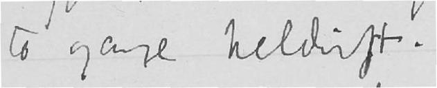to gange heldigt.
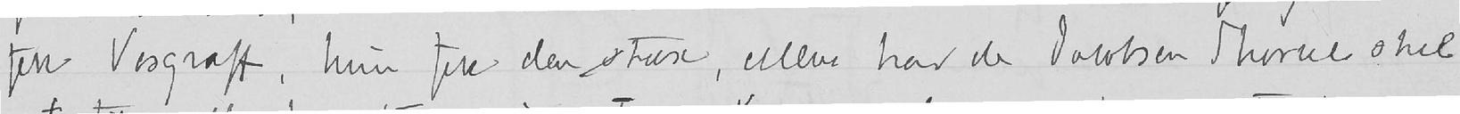frk Vosgraff, hun fik den strax, ellers har da Jacobsen Thorne skre-
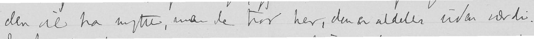den vil ha nytte, men de tror her, den er aldeles uden værdi.
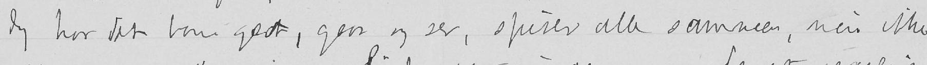Jeg har det bare godt, gaar og ser, spiser alle sammen, nei ikke
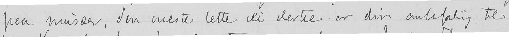paa musæer, den eneste lette vei dertil er din anbefaling til
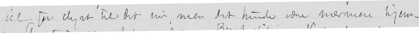vel for dyrt til det nu, men det kunde være nærmere hjem-
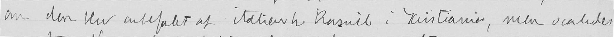om den blev anbefalet af italiensk konsul i Kristiania, men saaledes
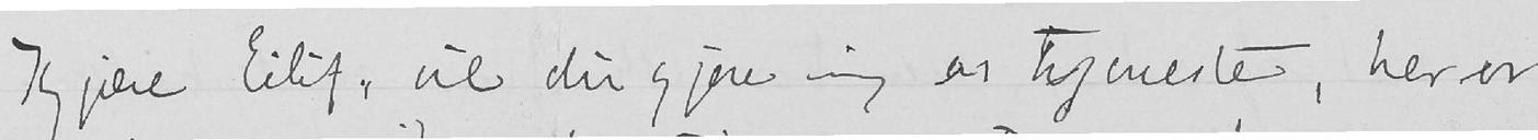Kjære Eilif, vil du gjøre mig en tjeneste, her er
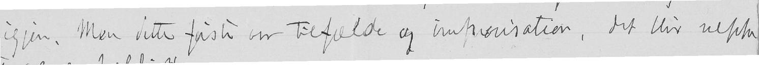igjen. Men dette første var tilfælde og improvisation, det blir neppe
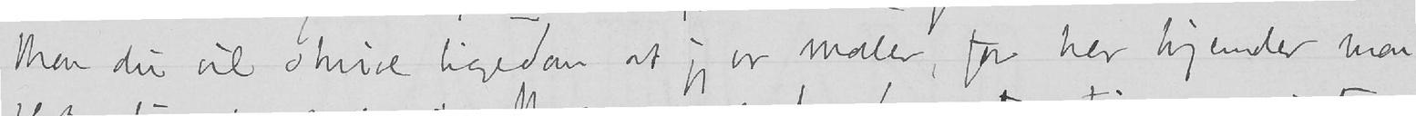Mon du vil skrive ligedan at jeg er maler, for her kjender man
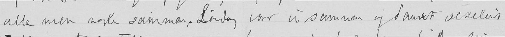alle men nogle sammen. Lørdag var vi sammen og danset vexelvis
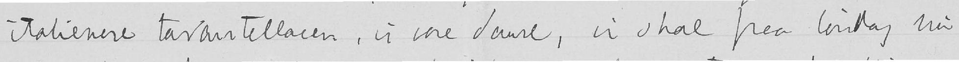italienere tarantellacen, vi vore danse, vi skal paa lørdag nu
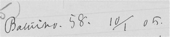Babuino 58. 10/1 05.
